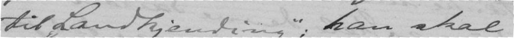til "Landkjending"; han skal
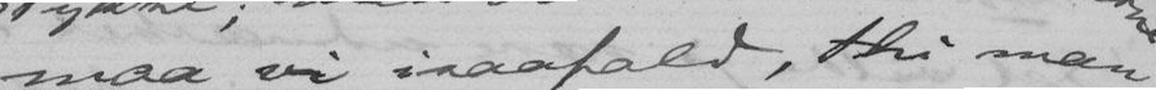maa vi isaafald, thi man
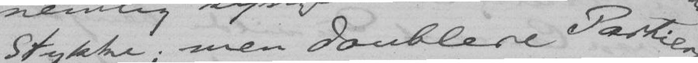stykke; men doublere Partierne
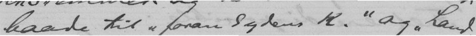baade til "foran Sydens K." og "Land-
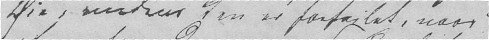Øie, medens den er forfeilet, naar
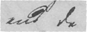end de
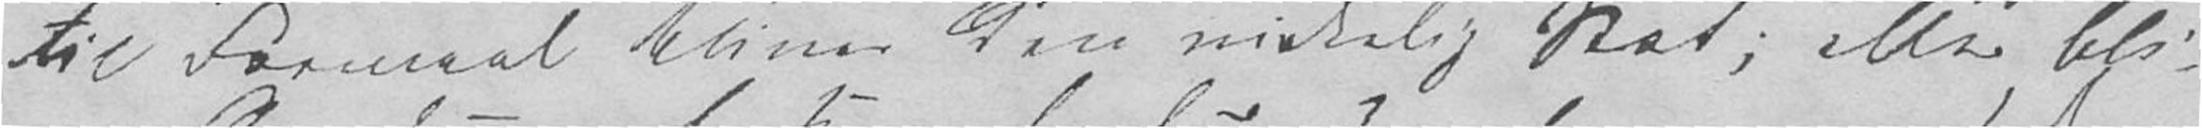til Formaal bliver den virkelig Stat; eller bli-
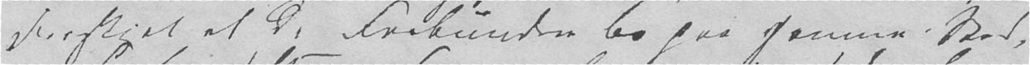Forskjel at de Forbundne bo paa samme Sted,
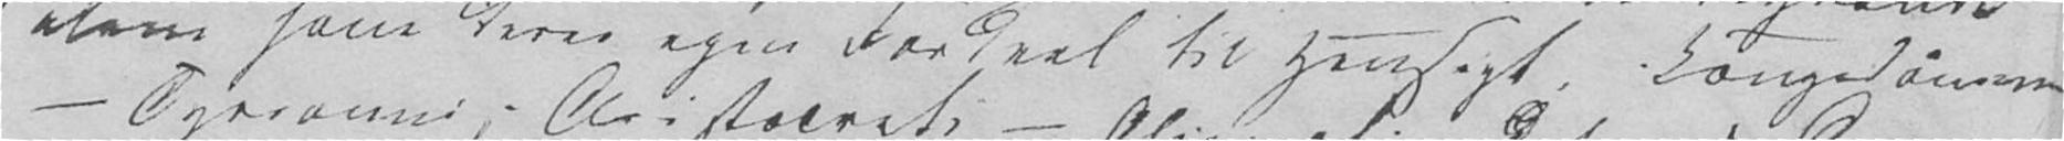alene have deres egen Fordeel til Hensigt. Kongedømme
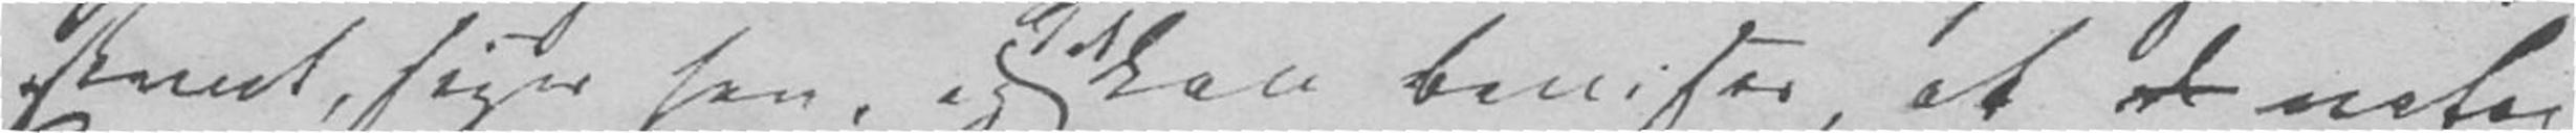stemt, siger han, og kan bevises, at de netop
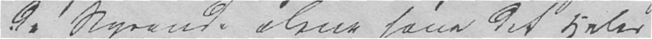de Styrende alene have det Heles
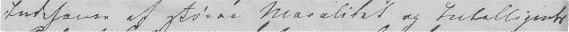Indehaver af større Moralitet og Intelligents
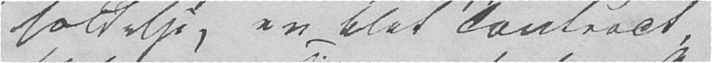holdelse, en blot Contract,
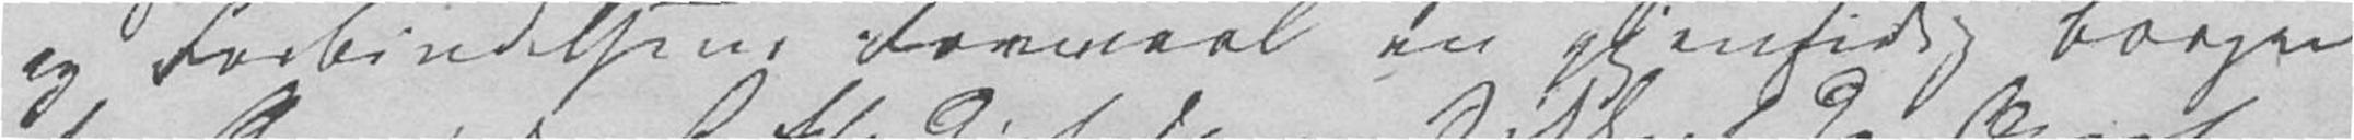og Forbindelsens Formaal en gjensidig Borgen
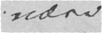være
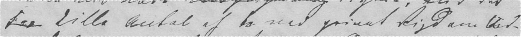faa lille Antal af de ved privat Rigdom Ud-
